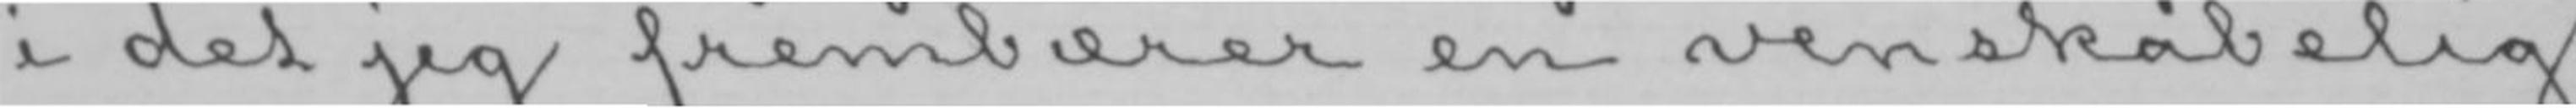i det jeg frembærer en venskabelig
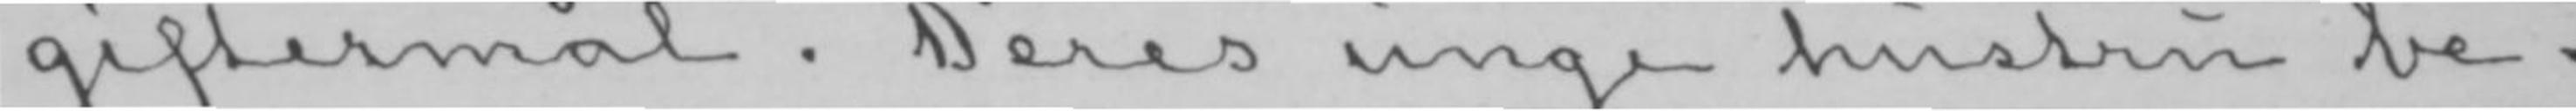giftermål. Deres unge hustru be-
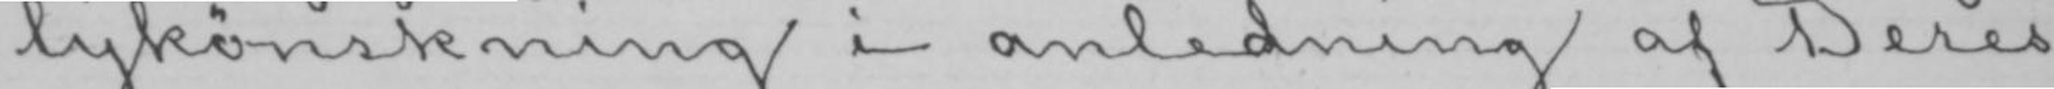lykønskning i anledning af Deres
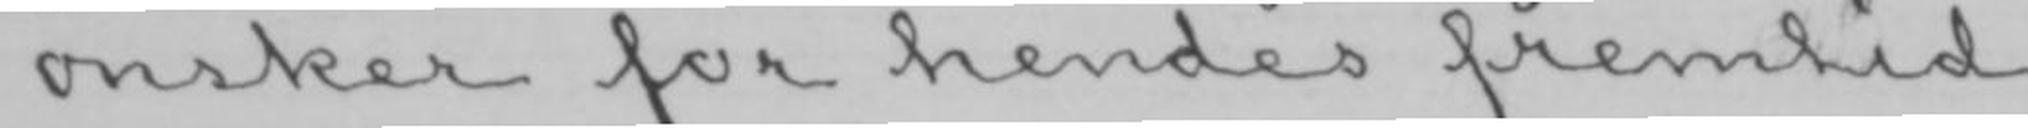ønsker for hendes fremtid.-
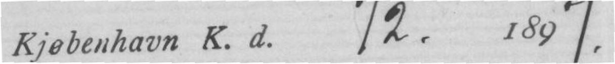Kjøbenhavn K. d. 5/2. 1897.
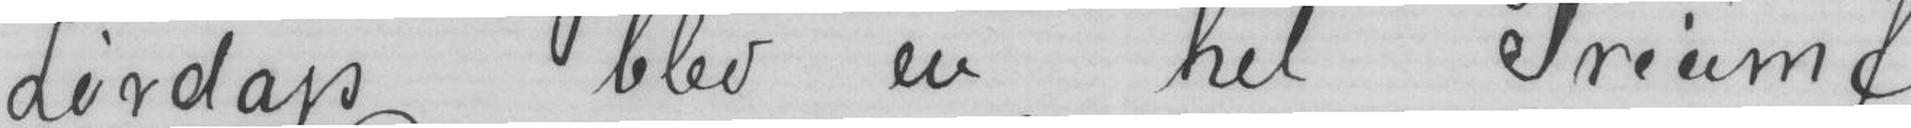Lordap blev en hel Triumf
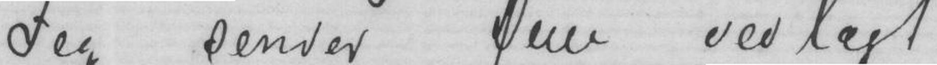Jeg sender Dem vedlagt
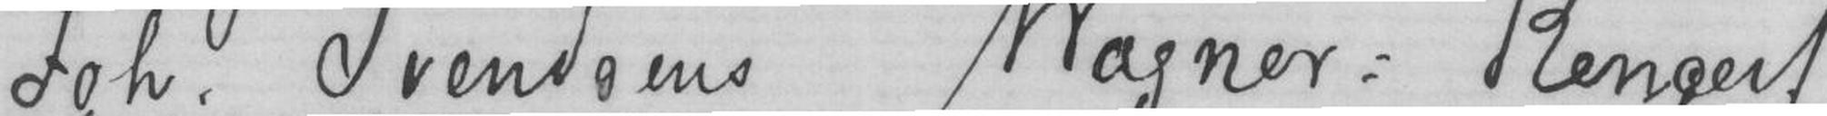Joh. Svendsens Wagner=Koncert
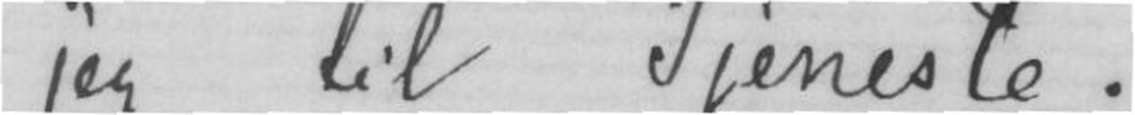jeg til Tjeneste.
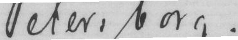Petersborg.
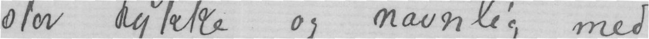stor Lykke og navnlig med
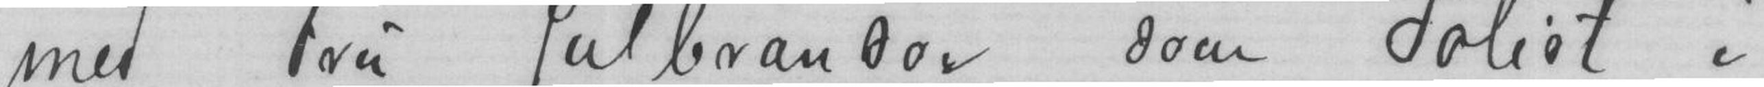med fru Gulbranson som Solist i
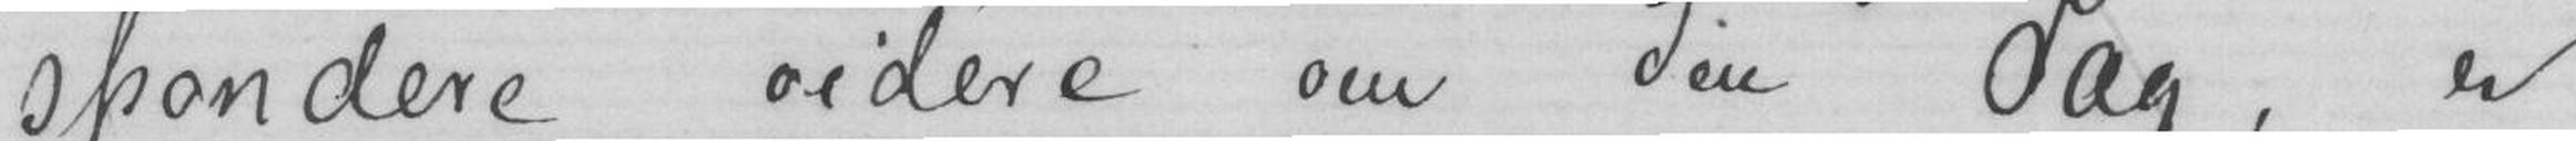spondere videre om den Sag, er
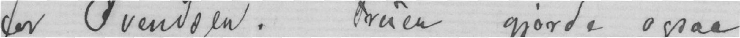for Svendsen. Fruen gjorde ogsaa
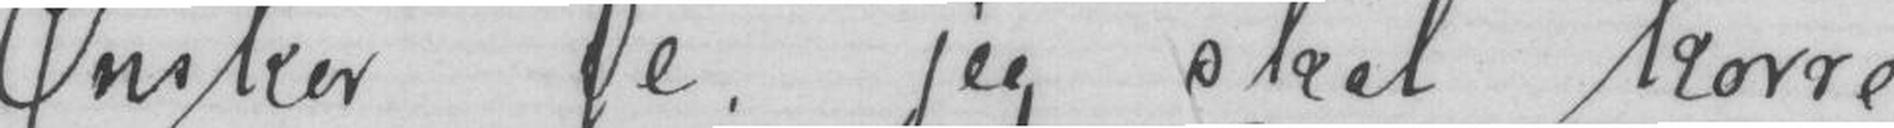Ønsker De jeg skal korre-
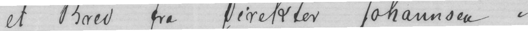et Brev fra Dirktør Johannsen i
